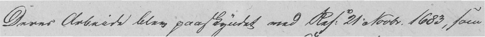Deres Arbeide blev paaskyndet med Res. 21. Novbr. 1683, som
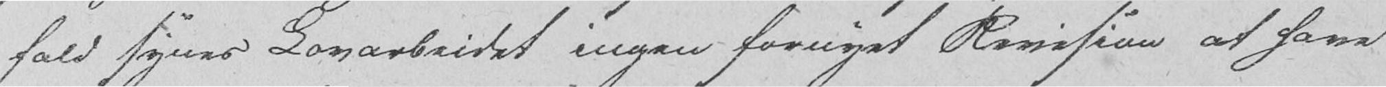fald synes Lovarbeidet ingen fornyet Revision at have
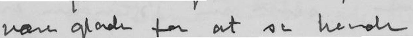være glade for at se hende.
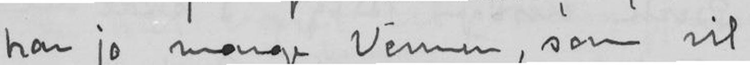har jo mange Venner, som vil
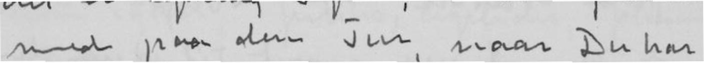med paa den Tur, naar Du har
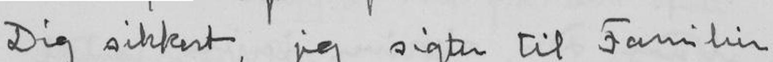Dig sikkert, jeg sigter til Familien
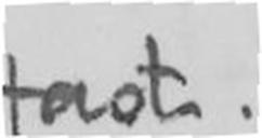fast.
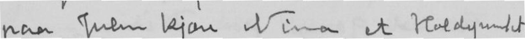paa Julen kjære Nina, et Holdepunkt
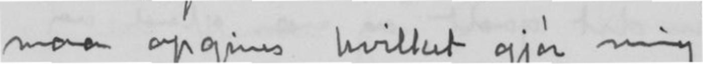maa opgives hvilket gjør mig
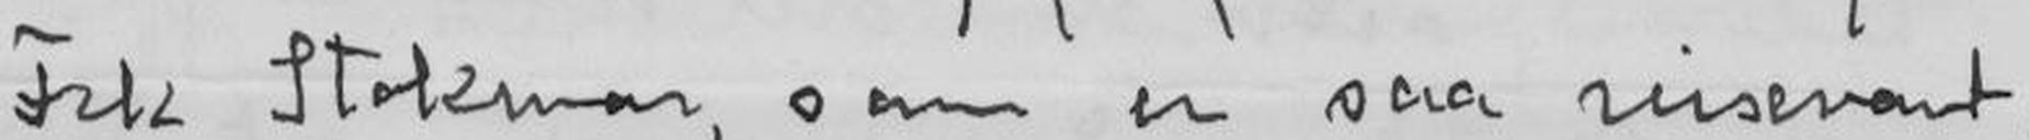Frk Stokman, som er saa reisevant.
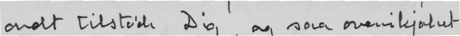ondt tilstøde Dig, og saa overikjølnet
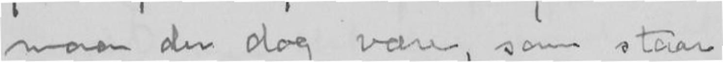maa der dog være, som staar
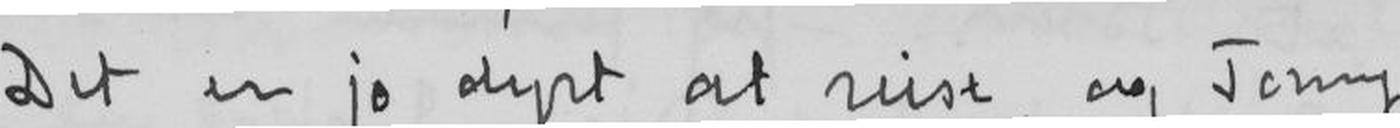Det er jo dyrt at reise, og Tonny
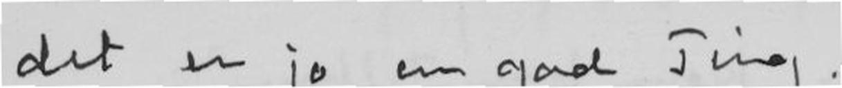det er jo en god Ting.
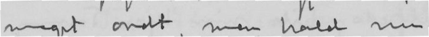meget ondt, men hold nu
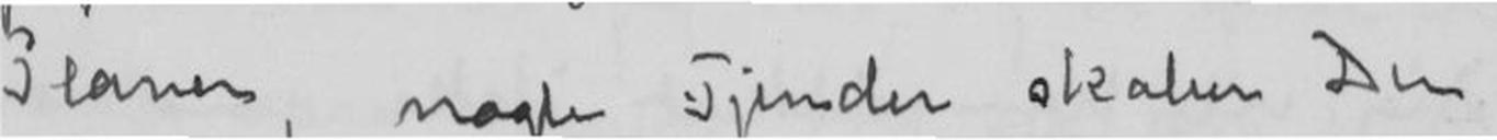Planer, nogle Fjender skaber Du
